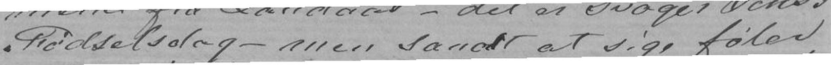Fødselsdag - men sandt at sige føler
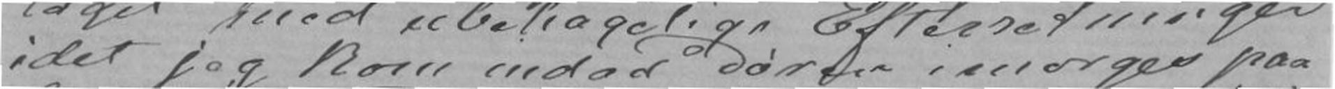idet jeg kom indad Døren imorgen paa
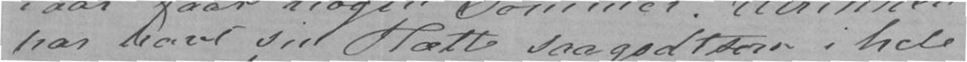har havt sin Hætte saagodtsom i hele
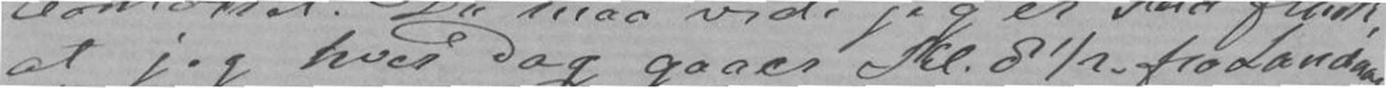at jeg hver Dag gaaer Kl.8½ fra Landaas
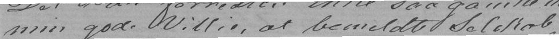min gode Villie, at bemeldte Selskab
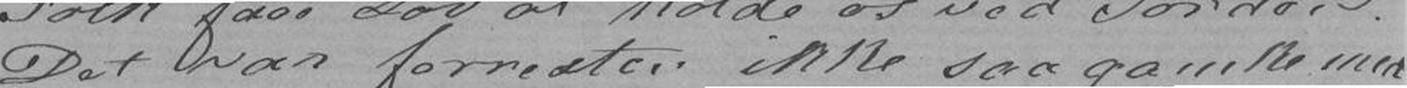Det var forresten ikke saa ganske med
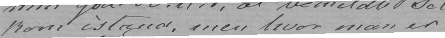kom istand, men hvor man er
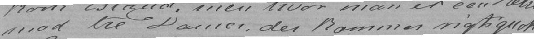mod tre Damer, der kommer rigtignok
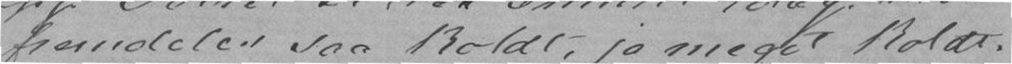fremdeles saa koldt, jo meget koldt.
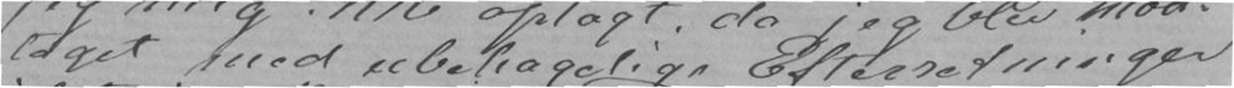taget med ubehagelige Efterretninger
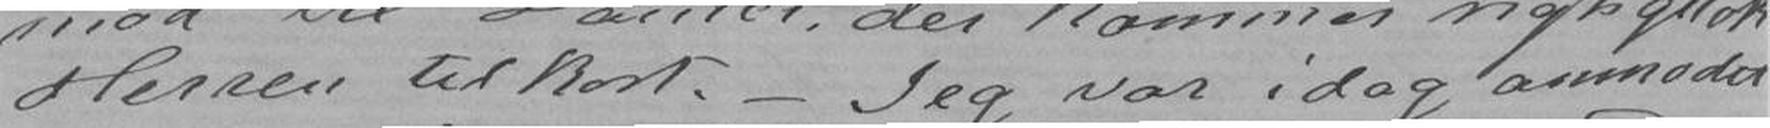Herren tilkort. - Jeg var idag anmodet
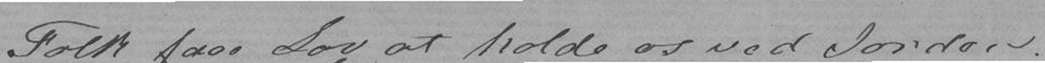Folk faae Lov at holde os ved Jorden
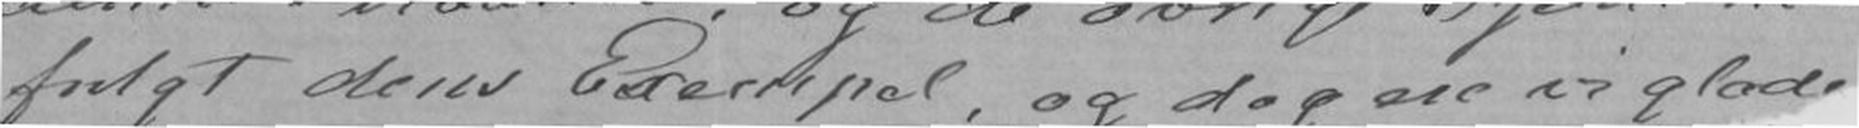fulgt dens Exempel, og dog ere vi glade
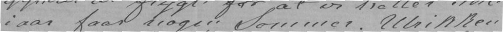iaar faar nogen Sommer. Ulrikken
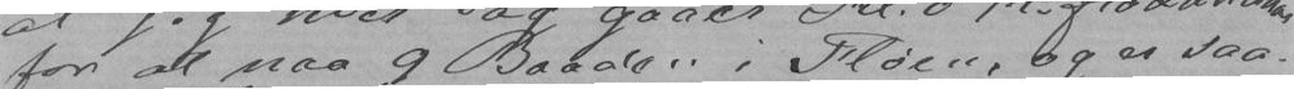for at naa 9 Baaden i Fløen, og er saa-
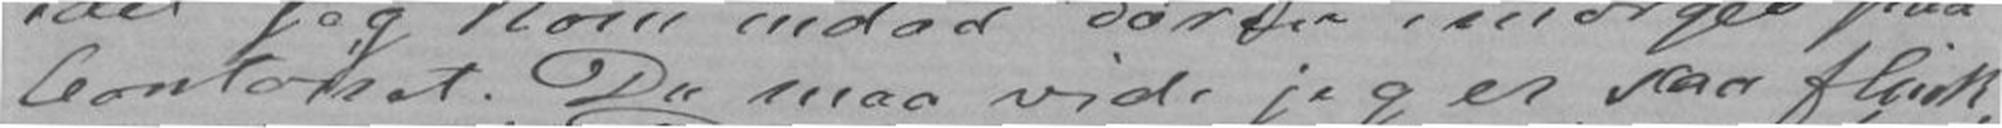Contoiret. Du maa vide jeg er saa flink,
